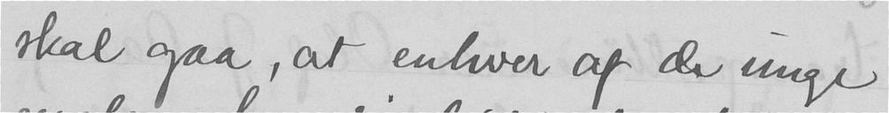skal gaa, at enhver af de unge
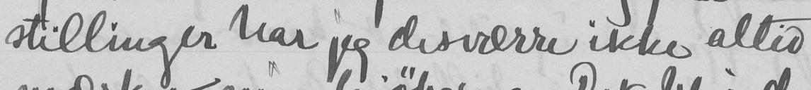stillinger har jeg desværre ikke altid
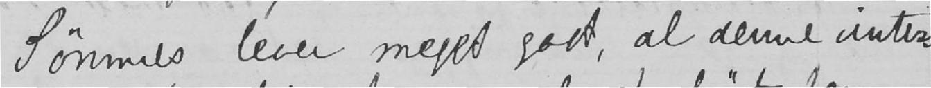Sømmes lever meget godt, al denne vinter-
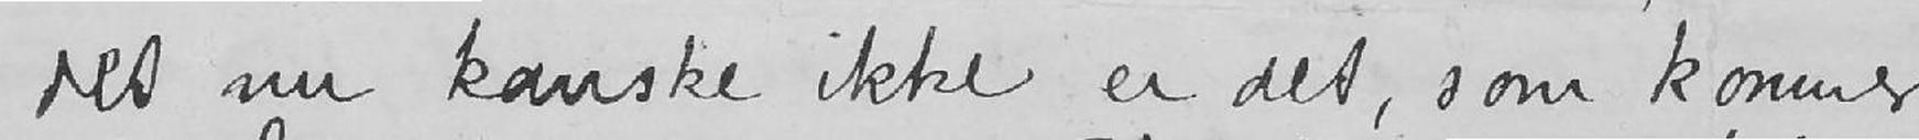det nu kanske ikke er det, som kommer
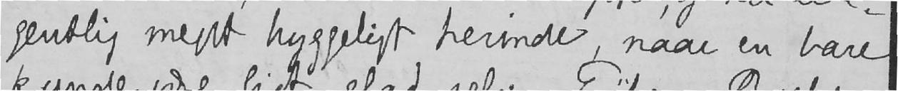gentlig meget hyggeligt herinde, naar en bare
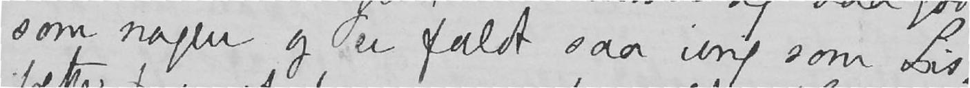som nogen og er fuldt saa ivrig som Lis-
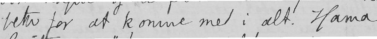beth for at komme med i alt. Hanna
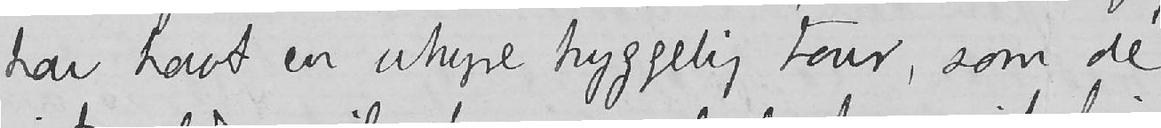har havt en uhyre hyggelig tour, som de
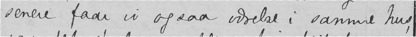senere faar vi ogsaa værelse i samme hus,
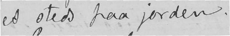et steds paa jorden.
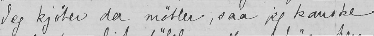Jeg kjøber da møbler, saa jeg kanske
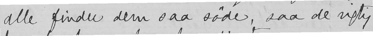alle finder dem saa søde, saa de rigtig
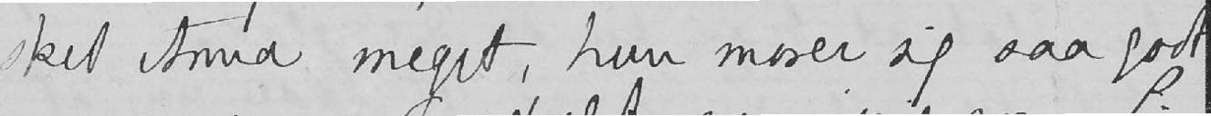ket Anna meget, hun morer sig saa godt
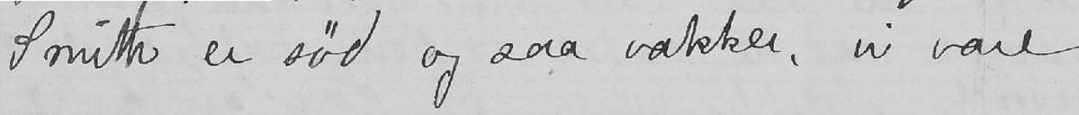Smith er sød og saa vakker, vi vare
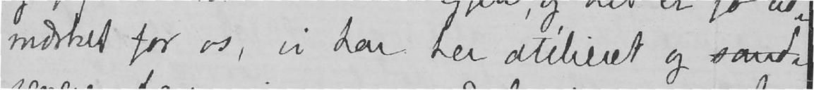mærket for os, vi har her atelieret og sand
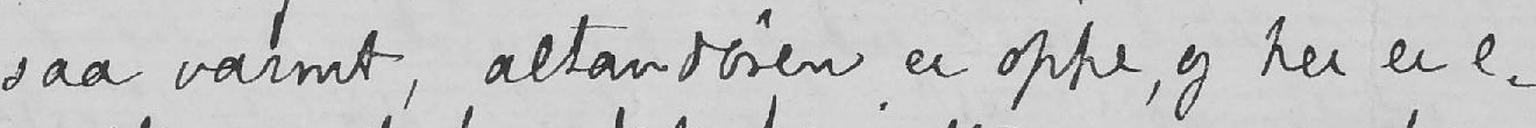saa varmt, altandøren er oppe, og her er e-
